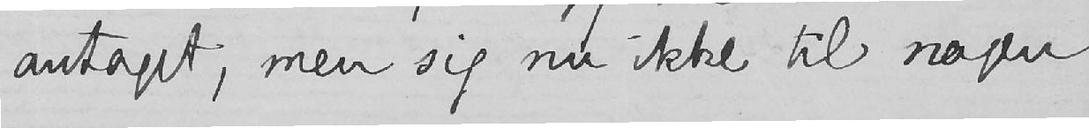antaget, men sig nu ikke til nogen
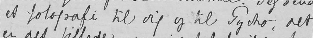et fotografi til dig til Tycho, det
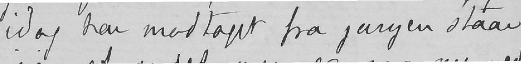idag har modtaget fra juryen staar
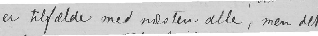er tilfælde med næsten alle, men det
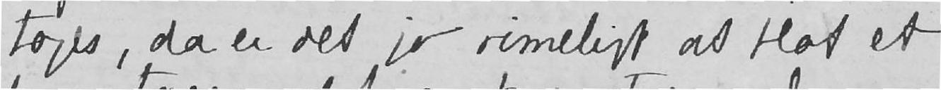tages, da er det jo rimeligt at blot et
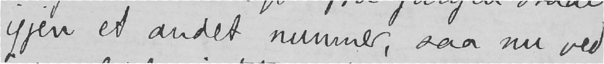igjen et andet nummer, saa nu ved
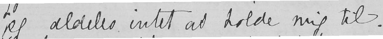jeg aldeles intet at holde mig til.
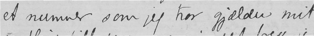et nummer som jeg tror gjælder mit
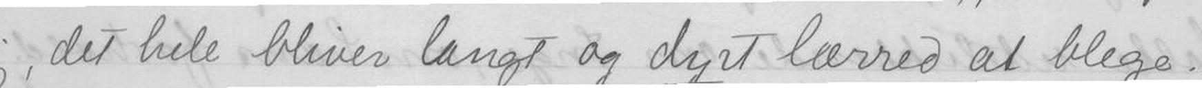jeg, det hele bliver langt og dyrt lærred at blege.
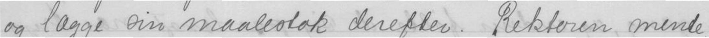og lægge sin maalestok derefter. Rektoren mente,
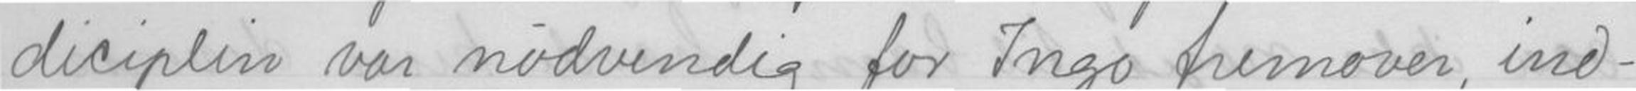diciplin var nødvendig for Ingo fremover, ind-
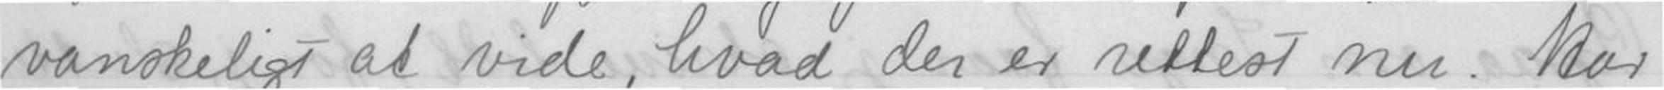vanskeligt at vide, hvad der er rettest nu: Har
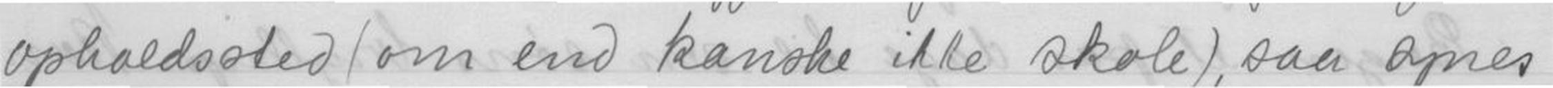opholdssted (om end kanske ikke skole), saa synes
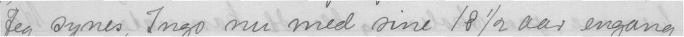Jeg synes, Ingo nu med sine 18 1/2 aar engang
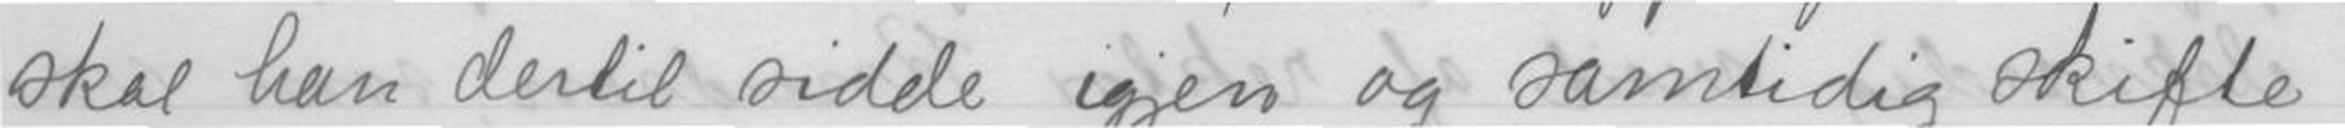skal han dertil sidde igjen og samtidig skifte
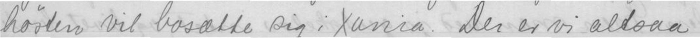høsten vil bosætte sig i Xania. Der er vi altsaa
